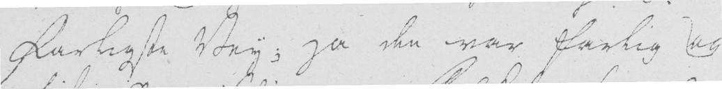Farligste Vey; Ja den var farlig og
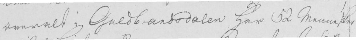overalt i Guldbrandsdalen har 52 Mennesker
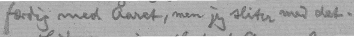færdig med Aaret, men jeg sliter med det.
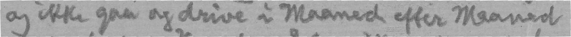og ikke gaa og drive i Maaned efter Maaned
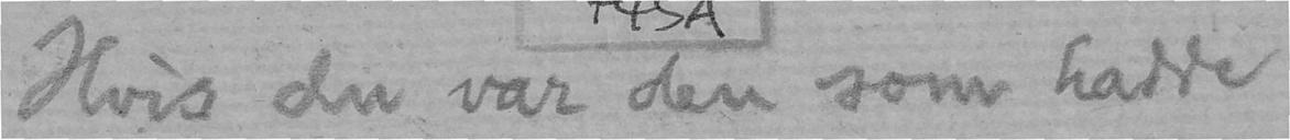Hvis du var den som hadde
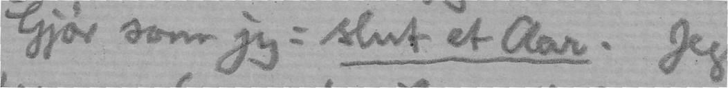Gjør som jeg: slut et Aar. Jeg
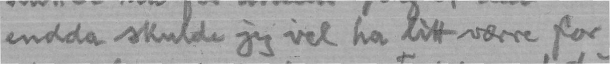endda skulde jeg vel ha litt værre for
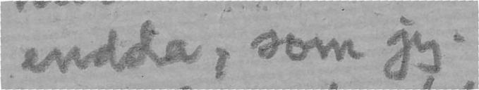endda, som jeg.
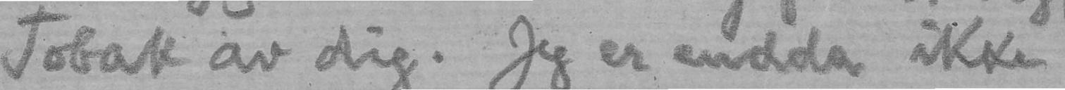Tobak av dig. Jeg er endda ikke
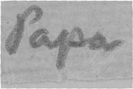Papa
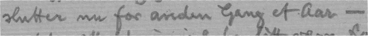slutter nu for anden Gang et Aar -
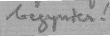begynder!
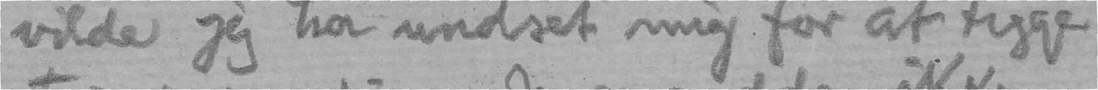vilde jeg ha undset mig for at tigge
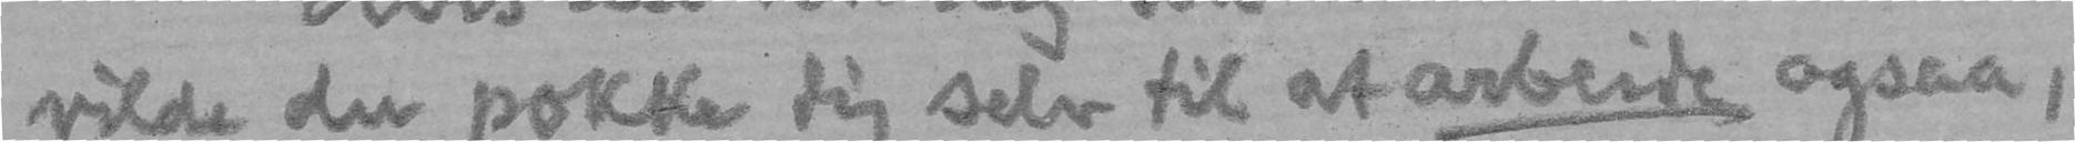vilde du pokke dig selv til at arbeide ogsaa,
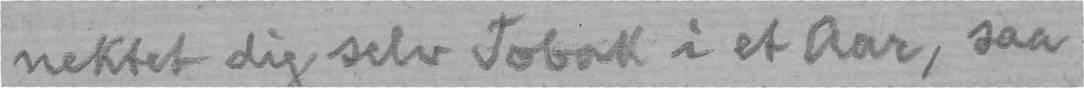nektet dig selv Tobak i et Aar, saa
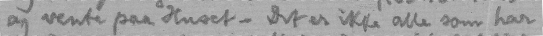og vente paa Huset. Det er ikke alle som har
